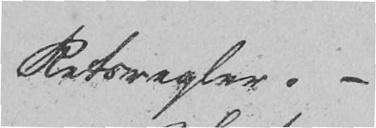Retsregler. –
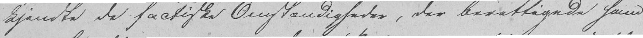kjendte de factiske Omstændigheder, der berettigede ham
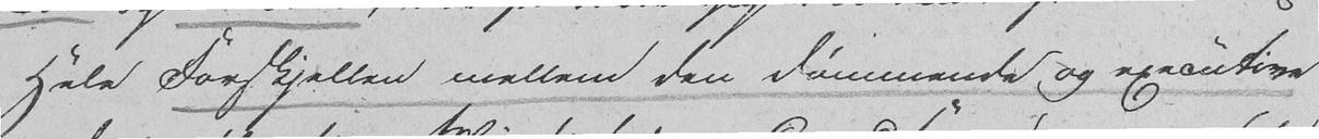Hele Forskjellen mellem den dømmende og executive
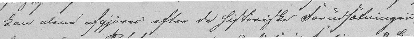kan alene afgjøres efter de historiske Forudsætninger,
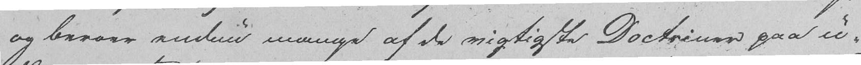og beroer endnu mange af de vigtigste Doctriner paa u-
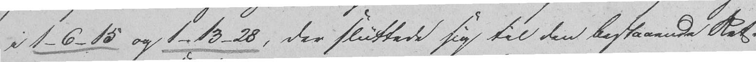i 1-6-15 og 1-13-28, der sluttede sig til den bestaaende Ret.
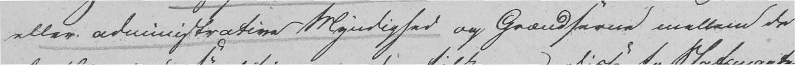eller administrative Myndighed og Grændserne mellem de
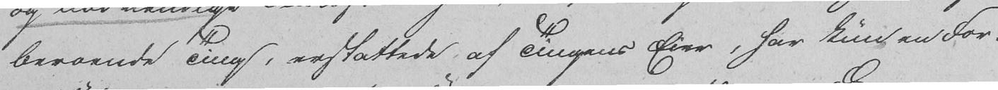beroende Ting, erstattede af Tingens Eier, har kun en For-
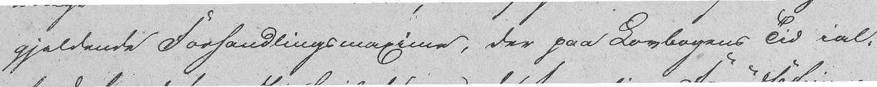gjeldende Forhandlingsmaxime, der paa Lovbogens Tid ial-
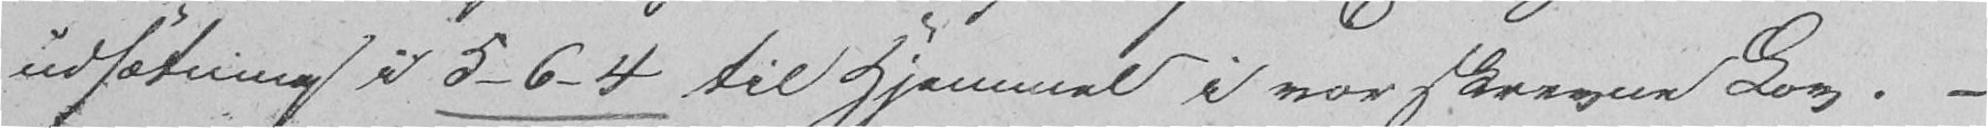udsætning i 5-6-4 til Hjemmel i vor skrevne Lov. -
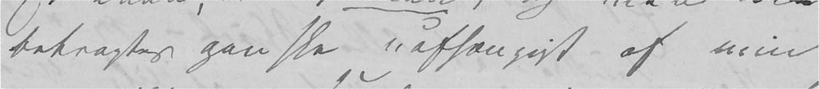betragtes ganske uafhængigt af min
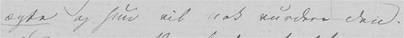ægte og hun vil nok vurdere den.
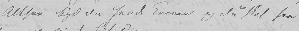Altsaa byd Du hende Kraven og Du skal see
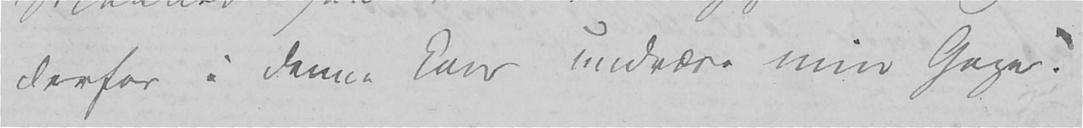derfor i denne kan undvære min Gage.
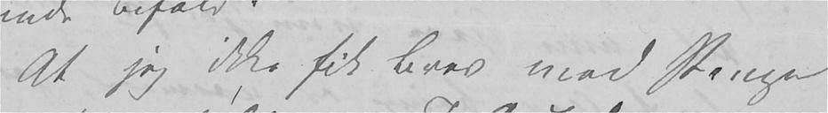At jeg ikke fik Brev med Penge
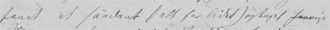faaet et saadant (alt for lidet) optaget forrige
